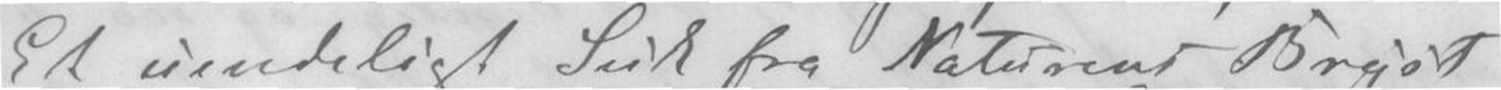Et uendeligt Suk fra Naturens Bryst
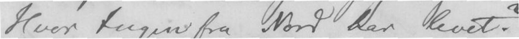Hvor ingen fra Nord har levet?
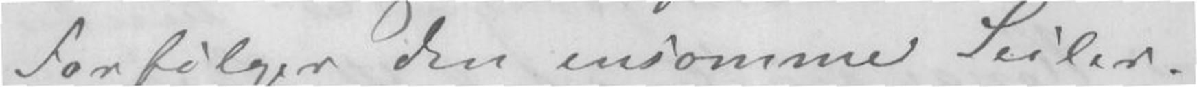som følger den ensomme Seiler.
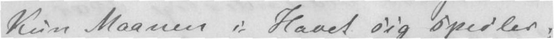Kun Maanen i, Havet sig speiler;
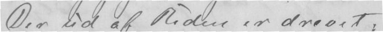Der ud af Beden er drevet;
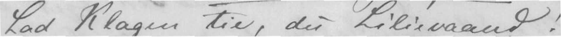Lad Klagen tie, du Lilievaand!
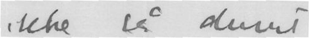ikke så drevet
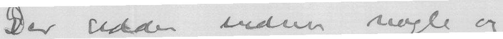Der sidder endnu nogle og
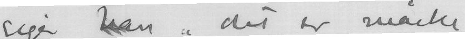siger han det er måske
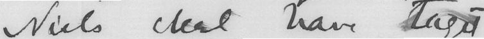Niels skal have taget
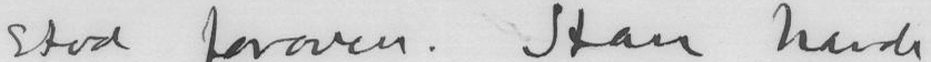stod foroven. Han havde
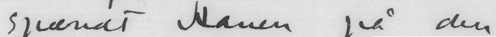spændt Hanen på den
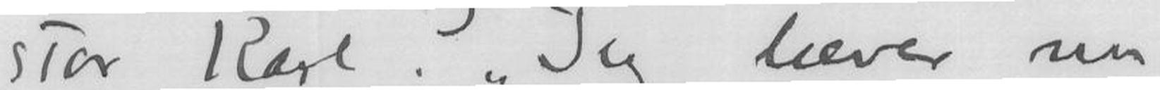stor Karl. Jeg læver nu
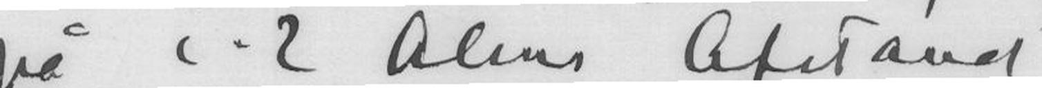på c. 2 Alens Afstand
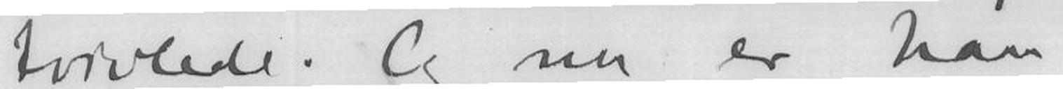tvivlede. Og nu er han
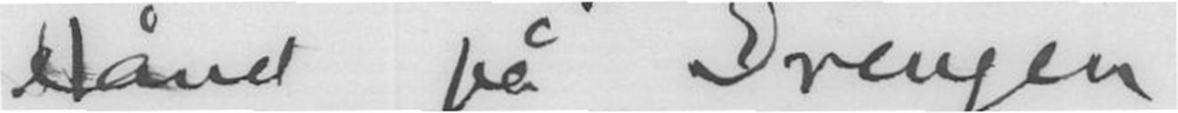Hånd på Drengen.
